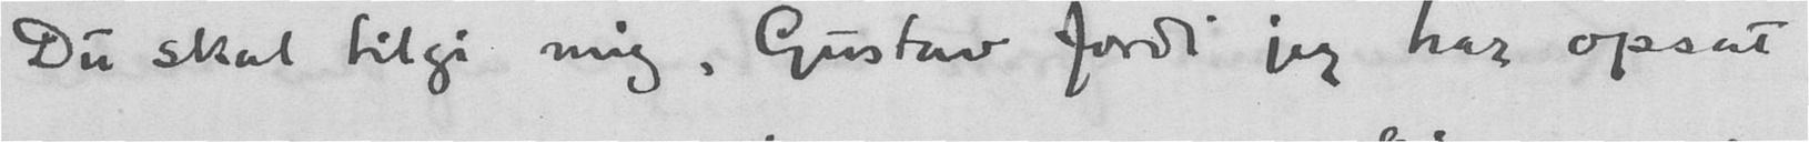Du skal tilgi mig, Gustav fordi jeg har opsat
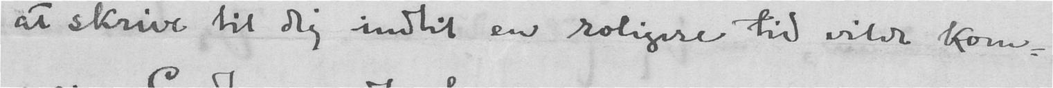at skrive til dig indtil en roligere tid vilde kom-
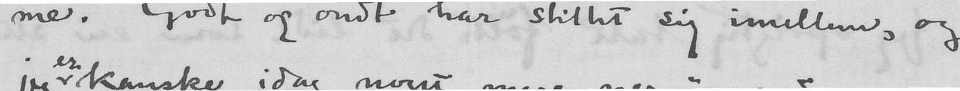me. Godt og ondt har stillet sig imellem, og
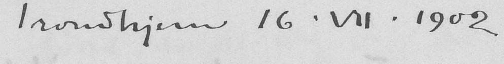Trondhjem 16.VII.1902
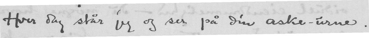Hver dag står jeg og ser på din aske-urne.
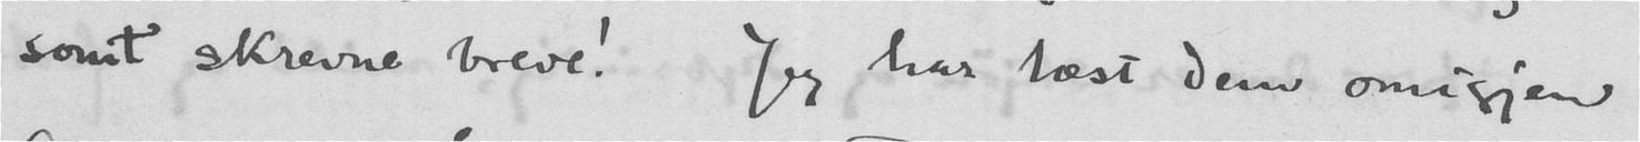somt skrevne breve! Jeg har læst dem omigjen
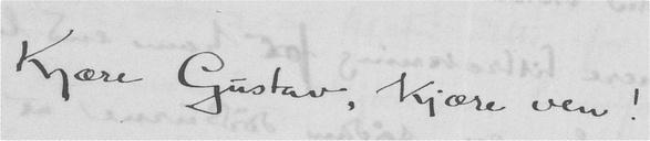Kjære Gustav, kjære ven!
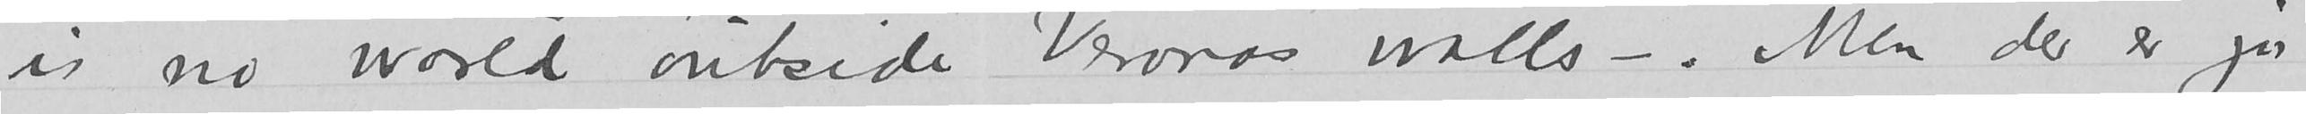is no world outside Veronas walls –. Men der er jo
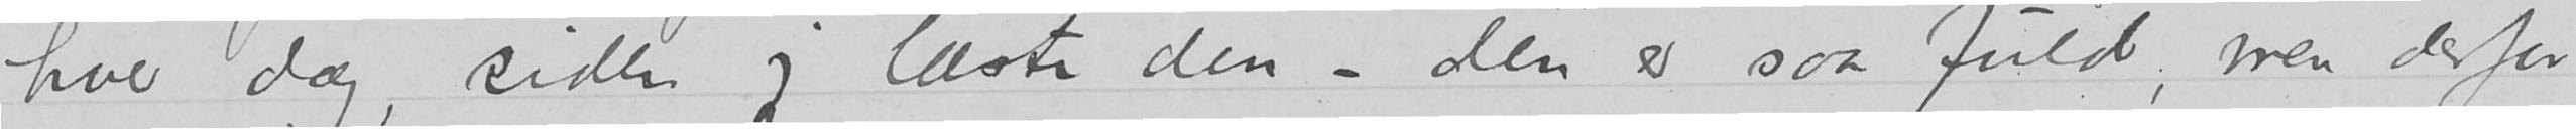siden jeg læste den – den er saa fuld, men derfor
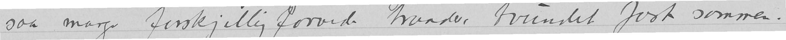saa mange forskjelligfarvede traader tvundet fast sammen.
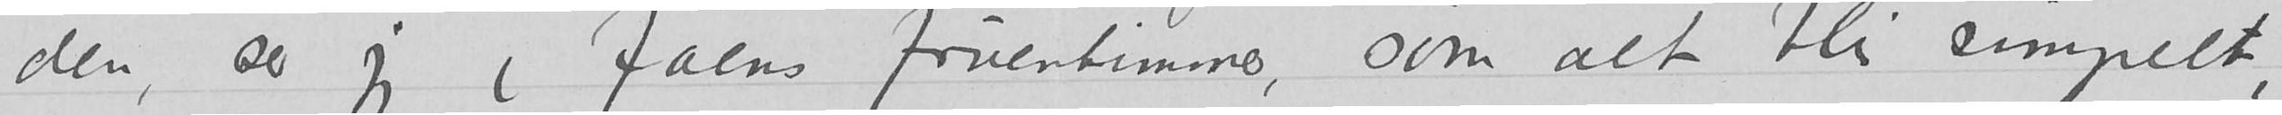den, ser jeg (faens fruentimmer, som alt blir simpelt,
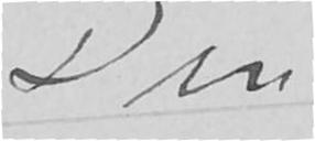Din
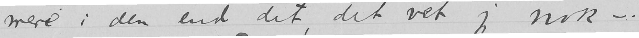mere i den end det, det vet jeg nok –.
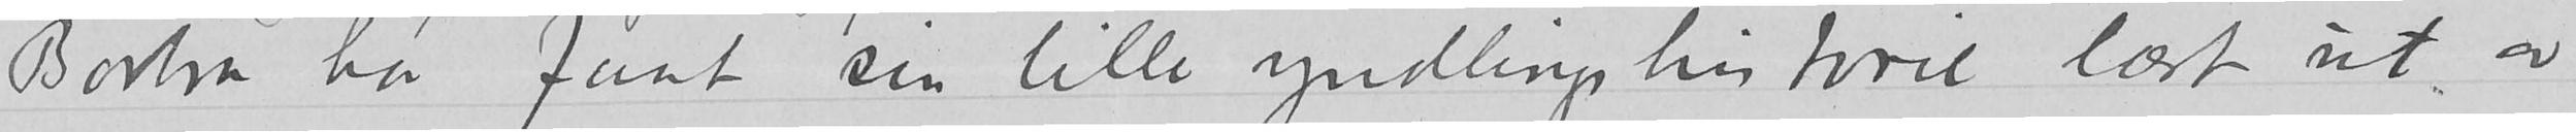Barbra har faat sin lille yndlingshistorie læst ut av
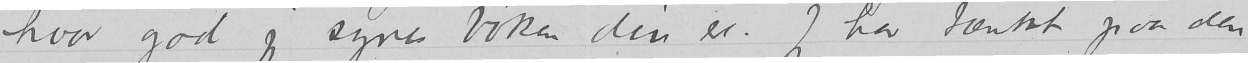hvor god jeg synes boken din er -  Jeg har tænkt paa den hver dag,
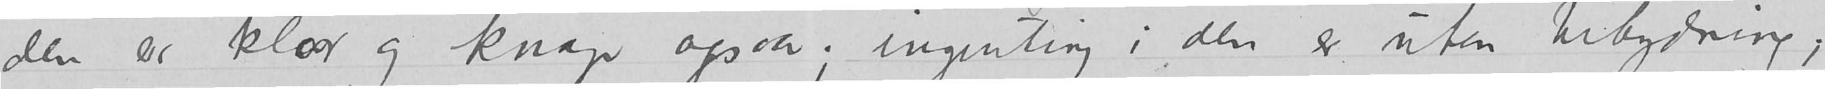den er klar og knap ogsaa; ingenting i den er uten betydning;
